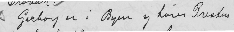Garborg er i Byen og hører Presten,
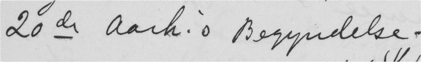20de Aarh.'s Begyndelse
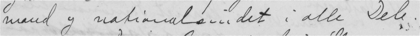mand og nationalsindet i alle Dele.
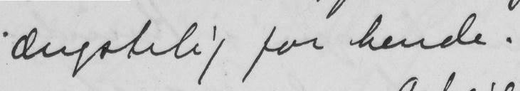ængstelig for hende.
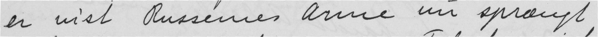er vist Russernes Arme nu sprængt
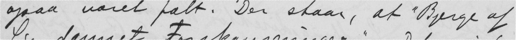ogsaa været fælt. Der staar, at "Bjerge af
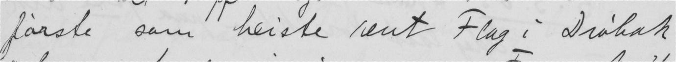første som heiste rent Flag i Drøbak
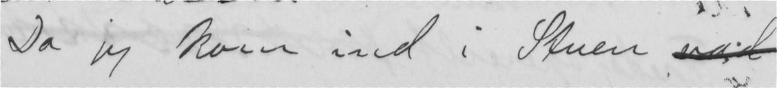Da jeg kom ind i Stuen
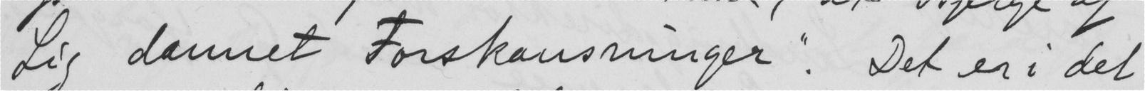Lig dannet Forskansninger". Det er i det
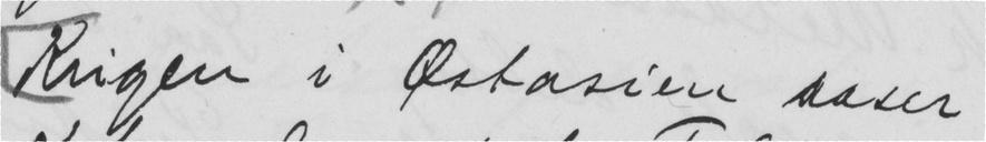Krigen i Østasien raser
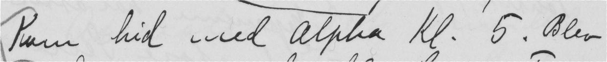Kom hid med Alpha Kl. 5. Blev
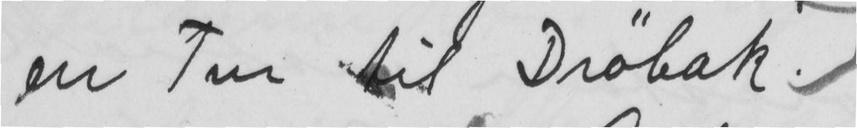en Tur til Drøbak
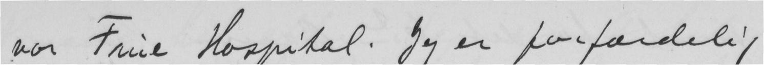vor Frue Hospital. Jeg er forfærdelig
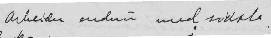Arbeider endnu med sidste
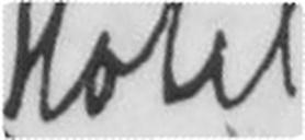Hotel
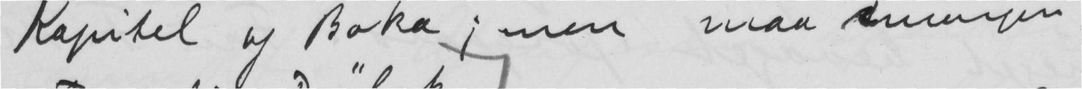Kapitel af Boka; men maa imogen
